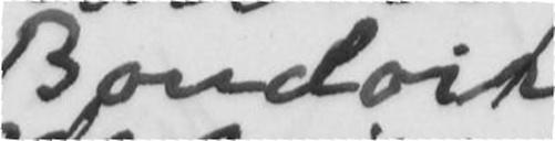Boudoir
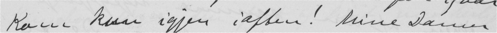Kom kun igjen iaften! Mine Damer
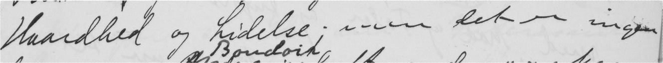Haardhed og Lidelse; men det er ingen
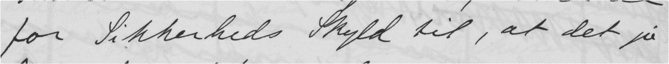for Sikkerheds Skyld til, at det jo
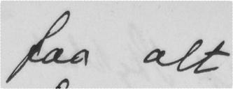faa alt
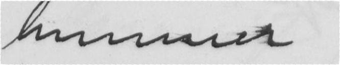lummer
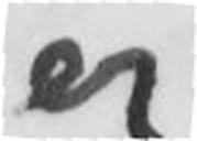er
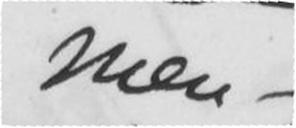Men
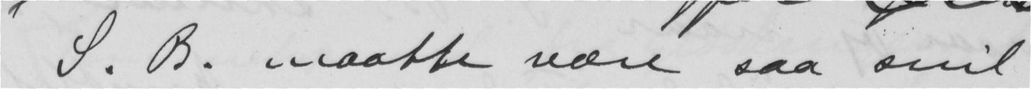S. B. maatte være saa snil
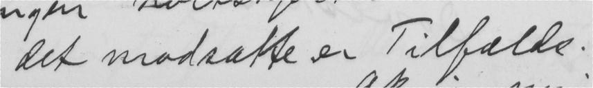det modsatte er Tilfælde.
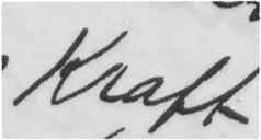Kraft
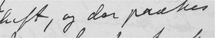luft, og der prækes
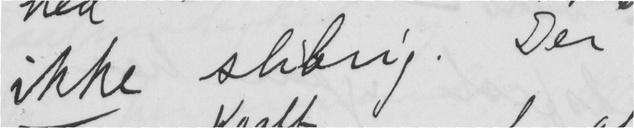ikke slibrig. Der
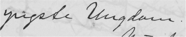yngste Ungdom.
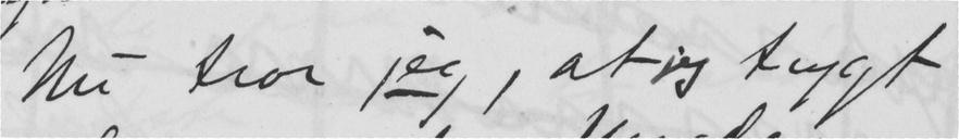Nu tror jeg, at jeg trygt
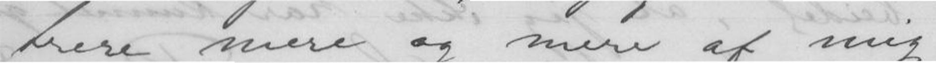trere mere og mere af mig
